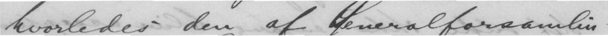hvorledes den af Generalforsamlin-
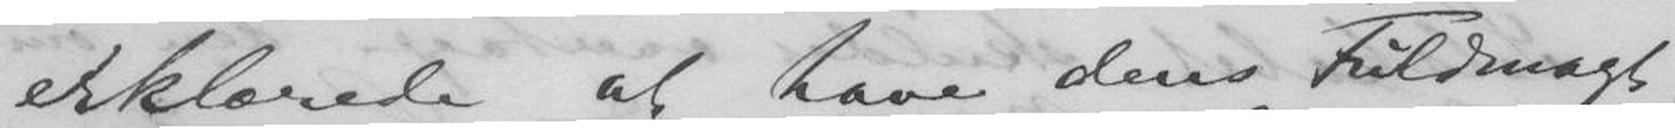erklærede at have dens Fuldmagt,
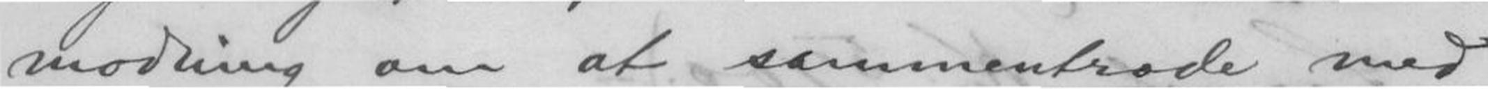modning om at sammentræde med
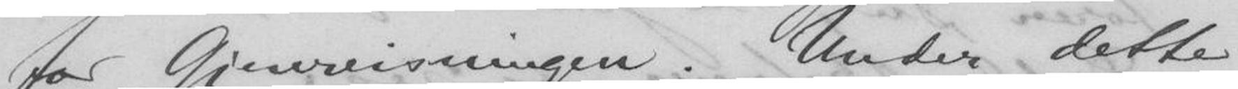for Gjenreisningen. Under dette
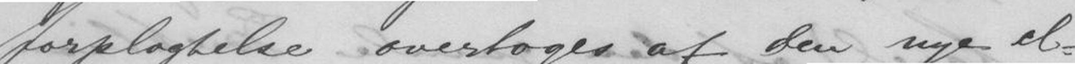forpligtelse overtoges af den nye el-
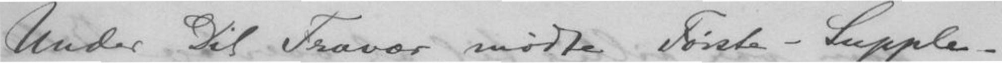Under Dit Fravær mødte Første-Supple-
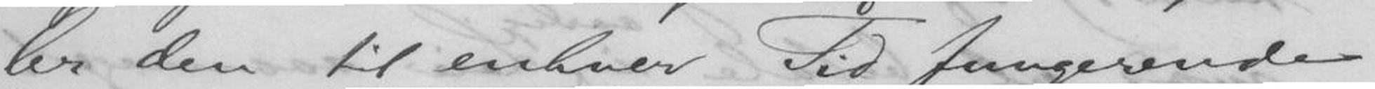ler den til enhver Tid fungerende
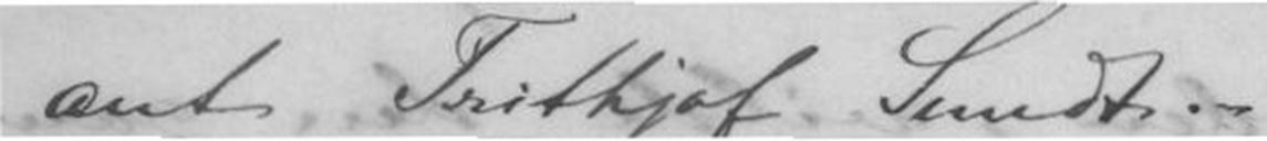ant Frithjof Sundt. -
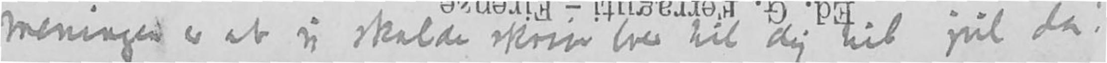Meningen er at jeg skulde skrive brev til dig til jul da!
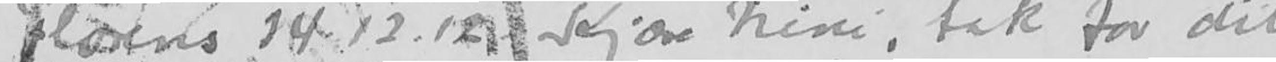Florens 14.12.12.	Kjære Nini, tak for dit
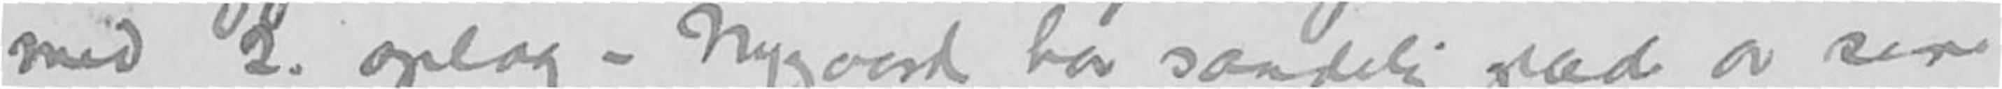med 2. oplag – Nygaard har sandelig glæde av sine
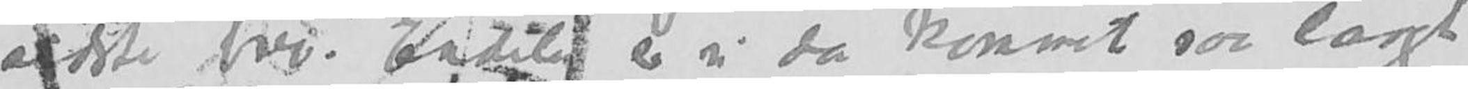sidste brev. Endelig er vi da kommet saa langt
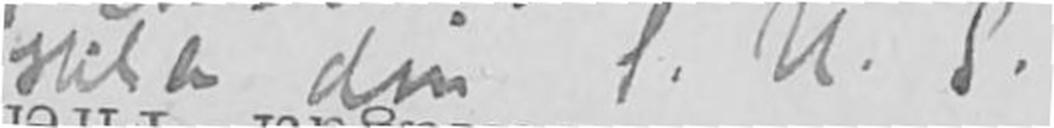Hilsen din S.U.S.
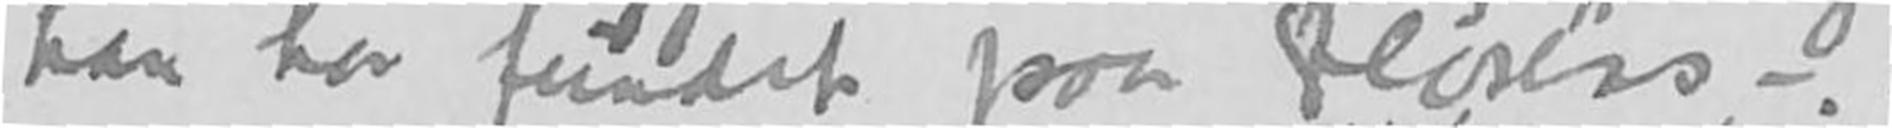han har fundet paa Florens –.
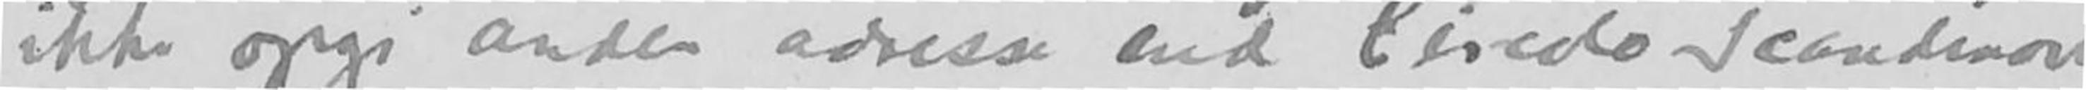ikke opgi anden adresse end Circolo Scandinavo
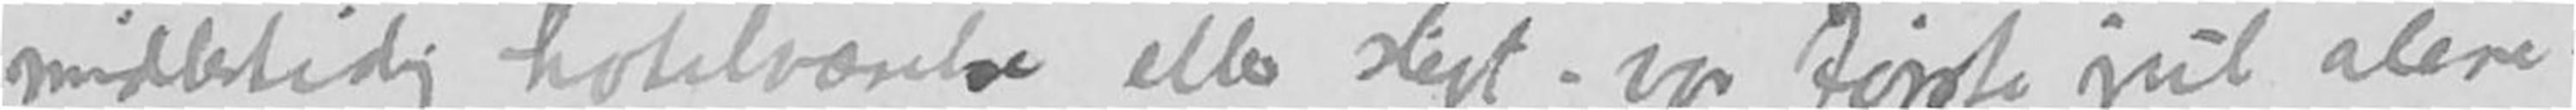midlertidig hotelværelse eller sligt – vor første jul alene –,
